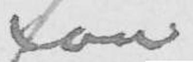for
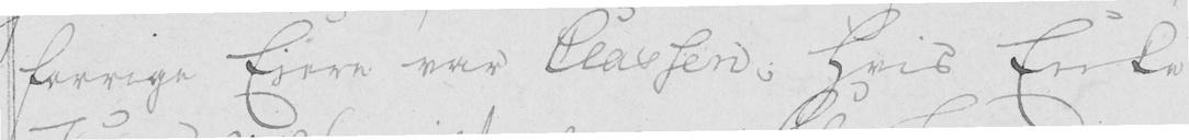forrige Eiere var Classen; hvis Enke
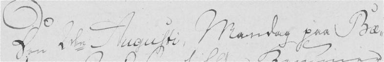Den 2den Augusti, Mandag paa Bæ-
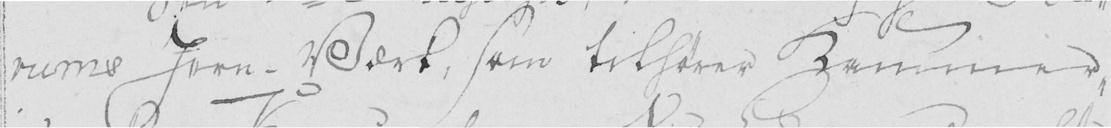rums Jern-Værk, som tilhører Kammer-
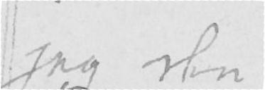jeg den
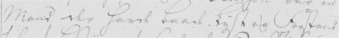Mand der havde baade Lyst og Forstand
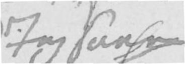Jeg saae
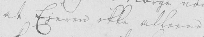at Ejeren ikke alleene
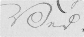Ved
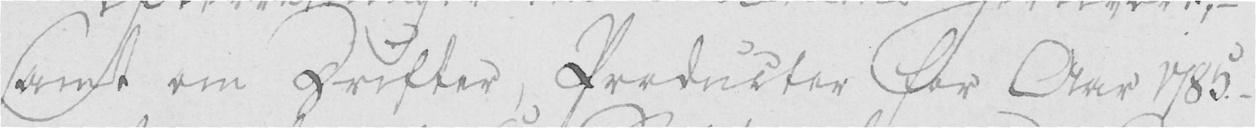samt om Drifter, Producter for Aar 1785. -
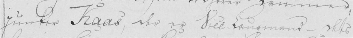Junker Kaas der er Vice-Laugmand - dets
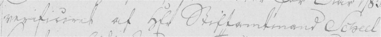verificeret af Hr Stiftamtmand Scheel
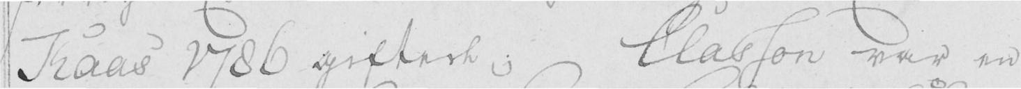Kaas 1786 giftede; Classon var en
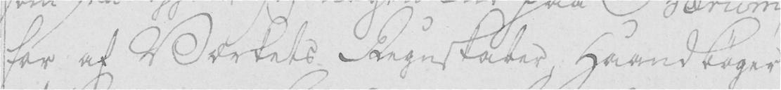for af Værkets Regnskaber, Haandbøger
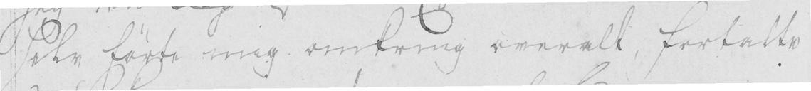selv førte mig omkring overalt, fortalte
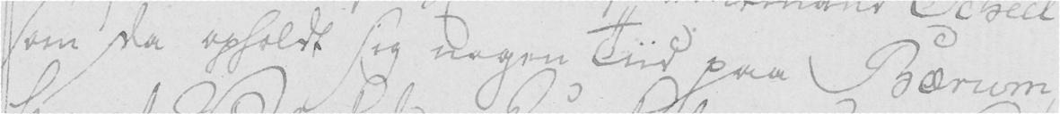som da opholdt sig nogen Tiid paa Bærum,
